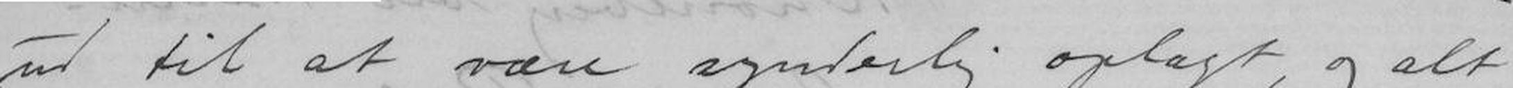ud til at være synderlig oplagt, og alt
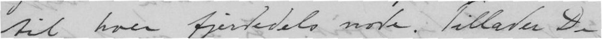til hver fjerdedels node. Tillader De
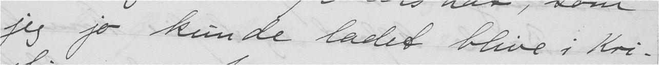jeg jo kunde ladet blive i Kri-
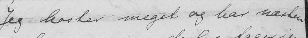Jeg hoster meget og har næsten
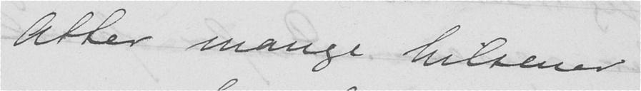Atter mange hilsener
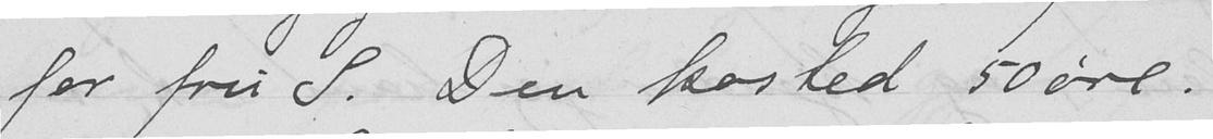for fru S. Den kosted 50 øre.
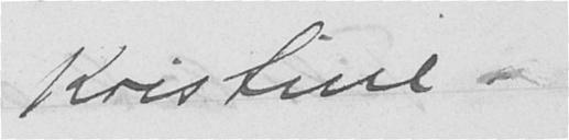Kristine.
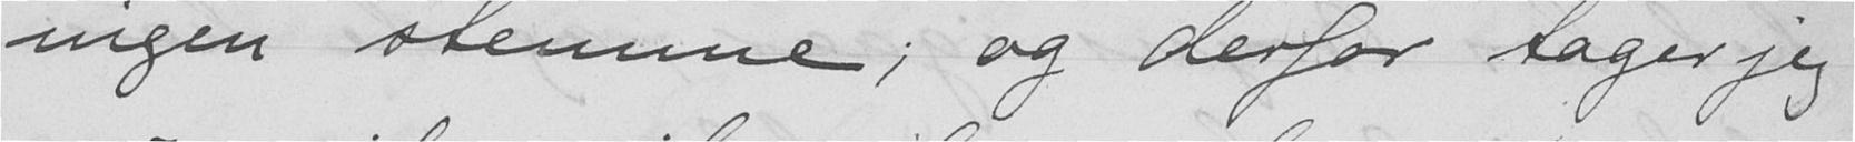ingen stemme; og derfor tager jeg
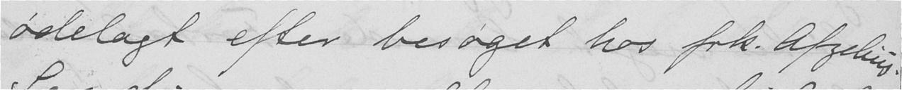ødelagt efter besøget hos frk. Afzelius.
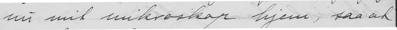nu mit mikroskop hjem, saa at
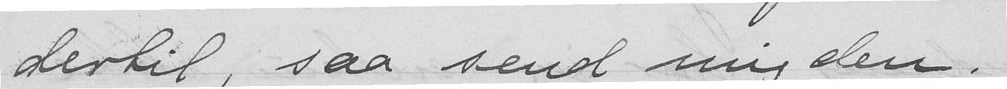dertil, saa send mig den.
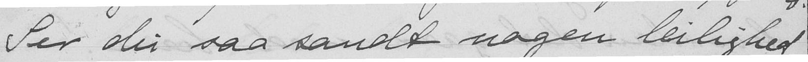Ser du saa sandt nogen leilighed
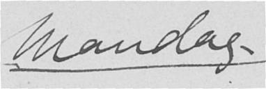Mandag
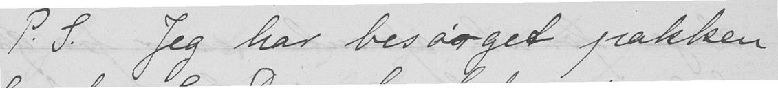P.S. Jeg har besørget pakken
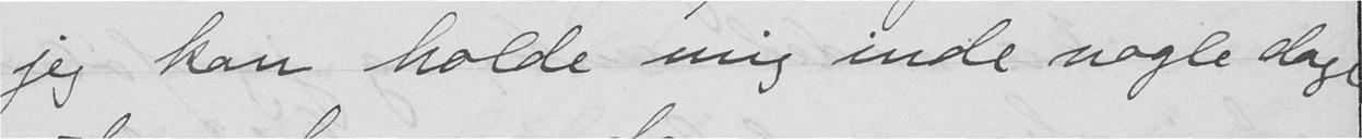jeg kan holde mig inde nogle dage
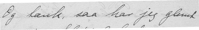Og tænk, saa har jeg glemt
